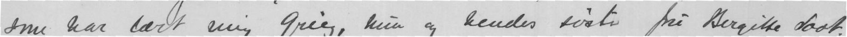som har lært mig Grieg, hun og hendes søster fru Bergitte Loot.
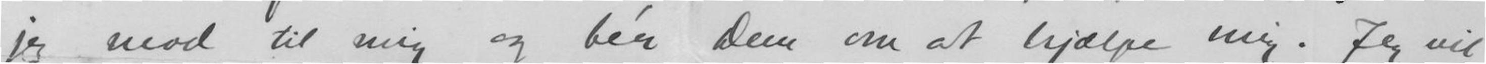jeg mod til mig og ber Dem om at hjælpe mig. Jeg vil
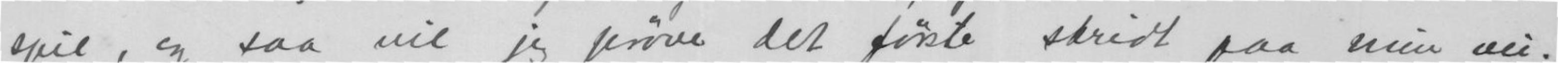spil, og saa vil jeg prøve det første skridt paa min vei.
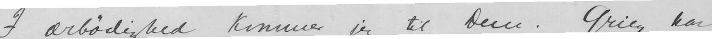I ærbødighed kommer jeg til Dem. Grieg har
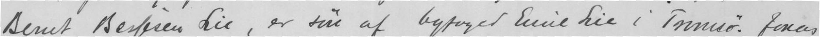Bernt Bestesen Lie, er søn af byfoged Emil Lie i Tromsø. Jonas
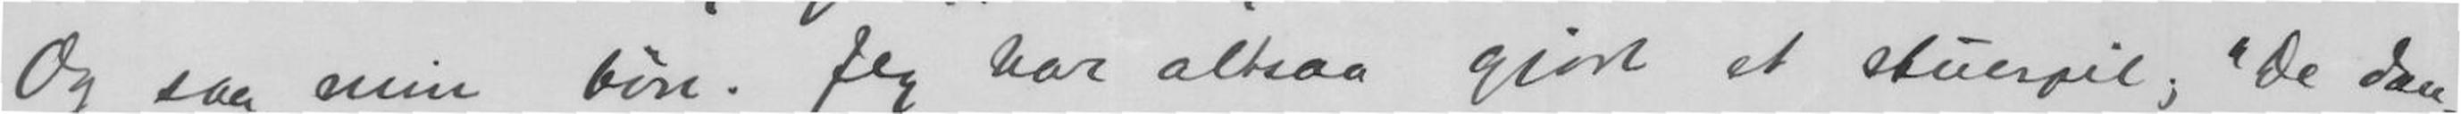Og saa min bøn. Jeg har altsaa gjort et skuespil; - "De dan-
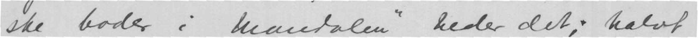ske boder i Mandalen" heder det; halvt
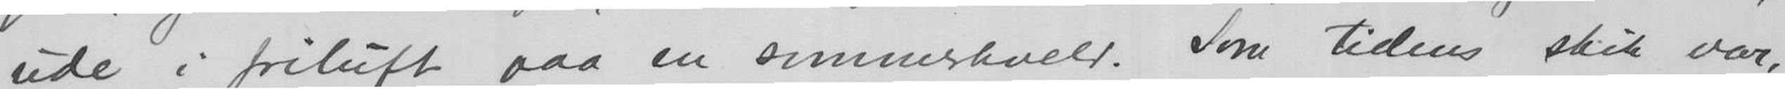ude i friluft paa en sommerkveld. Som tedens skik var,
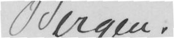Bergen.
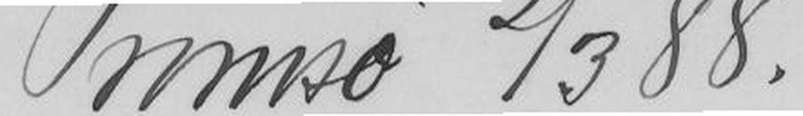Tromsø 2/3 88.
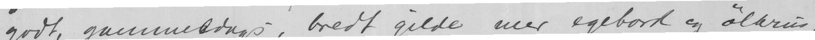godt, gammeldags, bredt gilde med egebord og ølkrus,
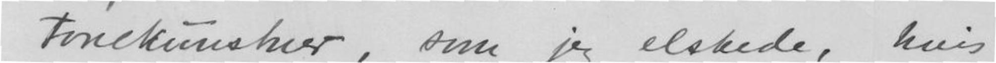Tonekunstner, som jeg elskede, hvis
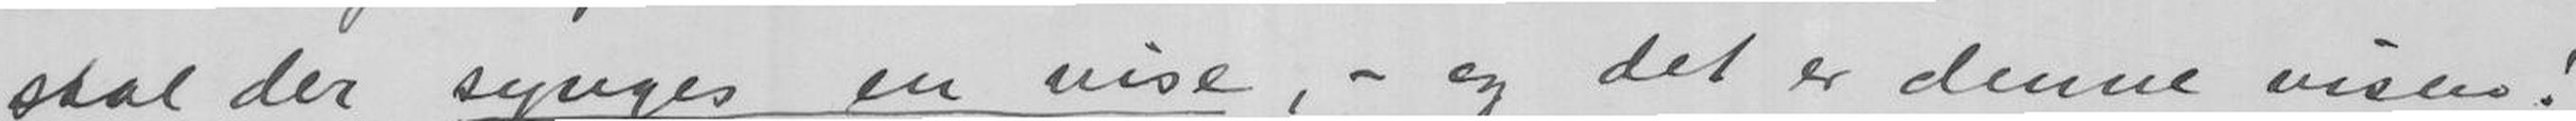skal der synges en vise, - og det er denne visen!
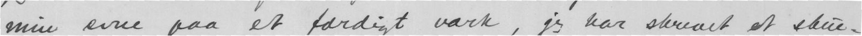mine ævne paa et færdigt værk, jeg har skrevet et skue-
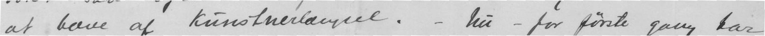at bæve af kunstnerlængsel. - Nu - for første gang tar
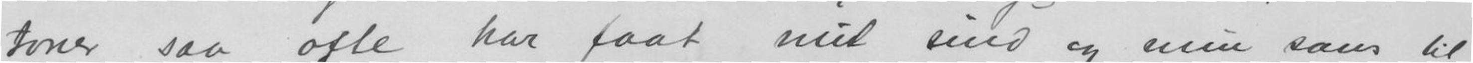toner saa ofte har faat mit sind og min sans til
